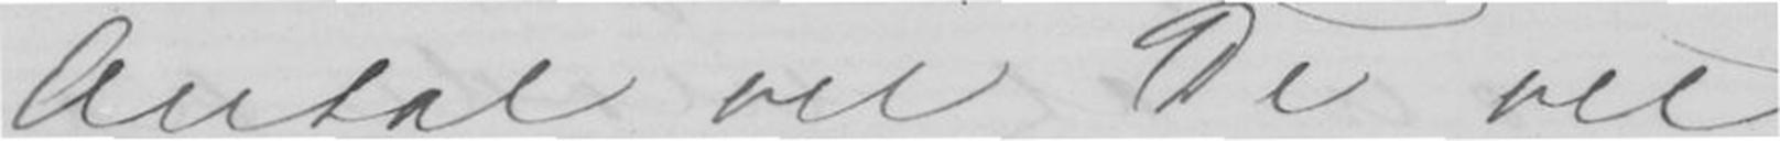Antal vil De vel
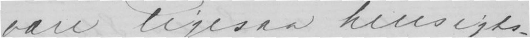være ligesaa hensigts-
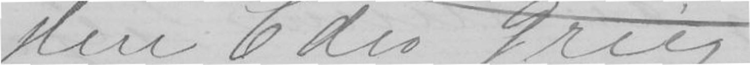Herr Eder Grieg
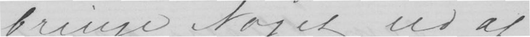bringe Noget ud af
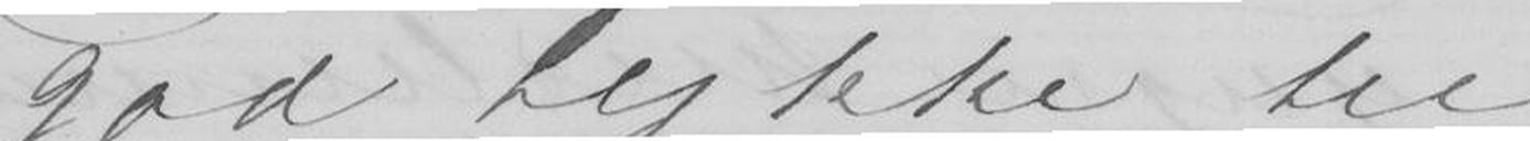god Lykke til
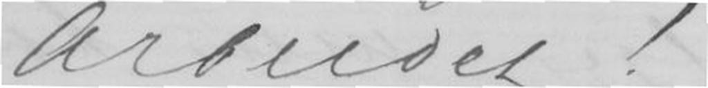Arbeidet
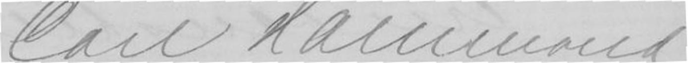Carl Hammond
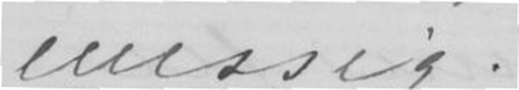messig.
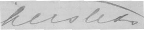hersteds
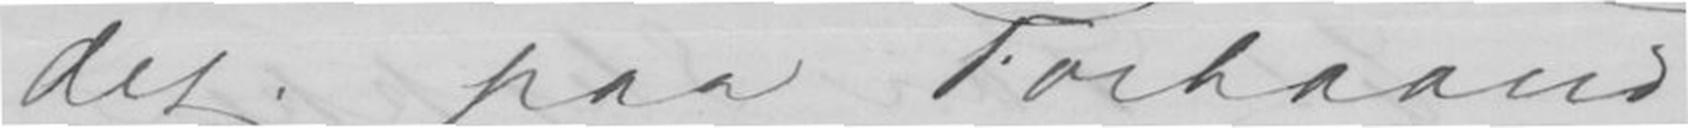det paa Forhaand
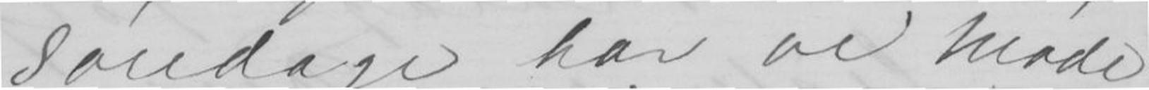Søndage har vi Møde
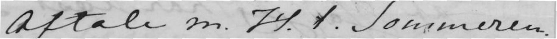Aftale m.H.t. Sommeren.
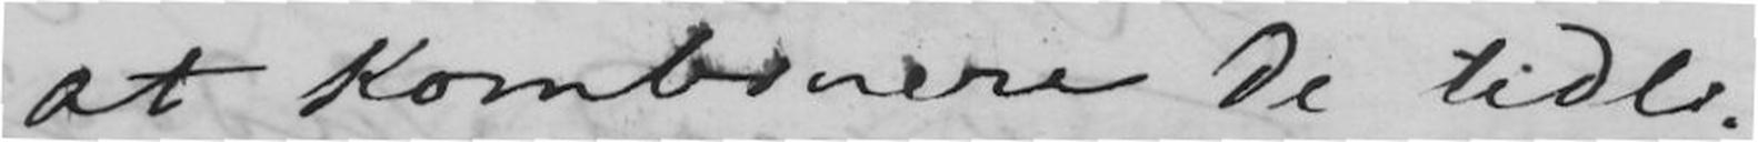at Kombinere De tidli-
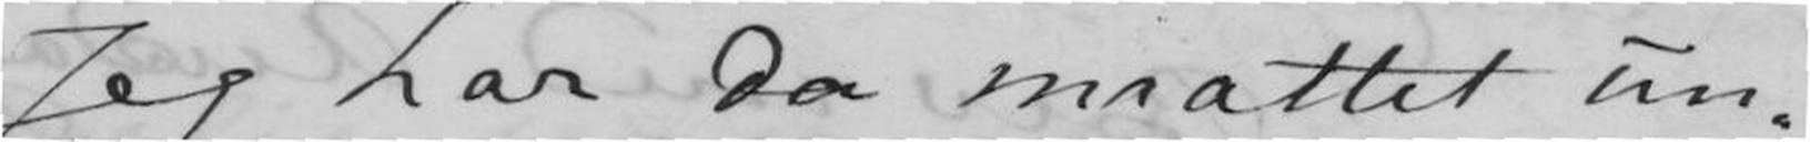Jeg har da maattet un-
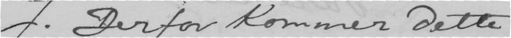J. Derfor Kommer Dette
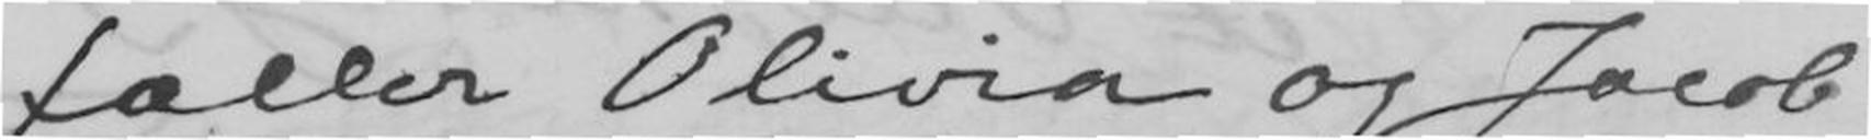fæller Olivia og Jacob
